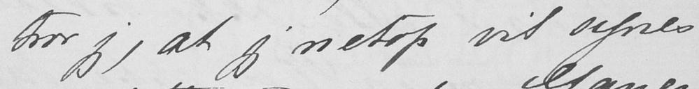tror jeg, at jeg netop vil synes
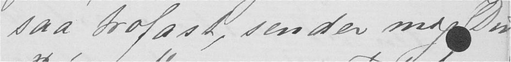saa trofast, sender mig Dine
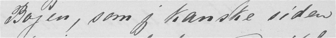Bogen, som jeg kanske siden
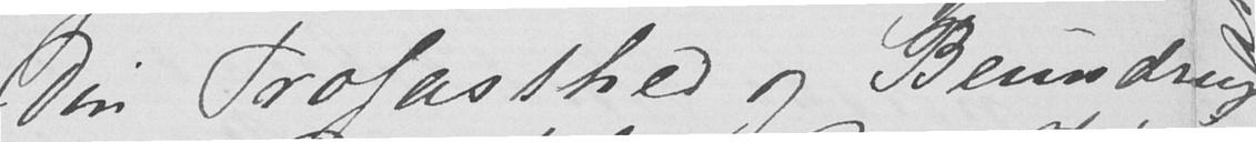Din Trofasthed og Beundring
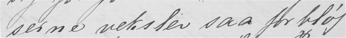serne veksler saa forbløf-
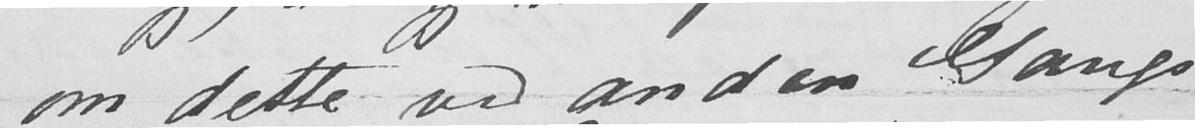om dette ved anden Gangs
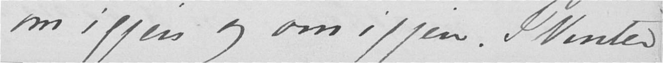om igjen og om igjen. I Vinter
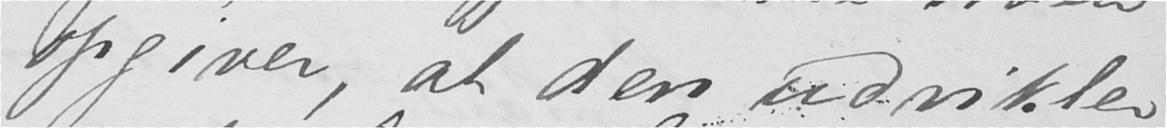opgiver, at den udvikler
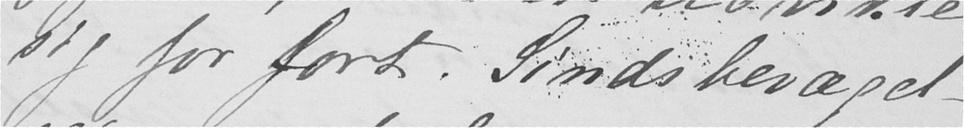sig for fort. Sindsbevægel-
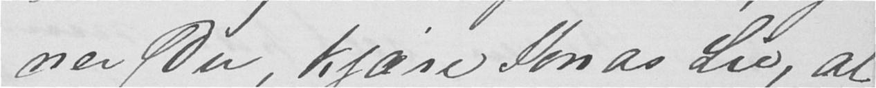ner Du, Kjære Jonas Lie, at
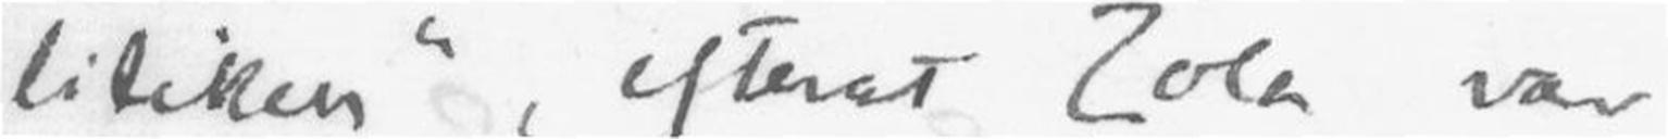litiken, efterat Zola var
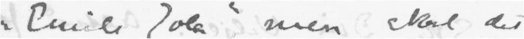Emile Zola, men skal der
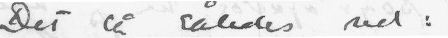Det så således ud:
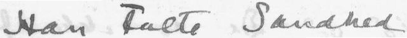Han Talte Sandhed.
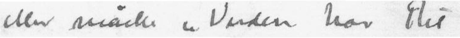eller måske Verden har Ret
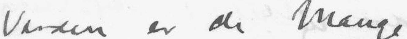Verden er de Mange.
